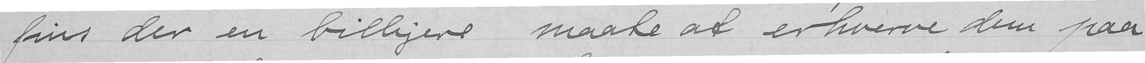fins der en billigere maate at erhverve dem paa
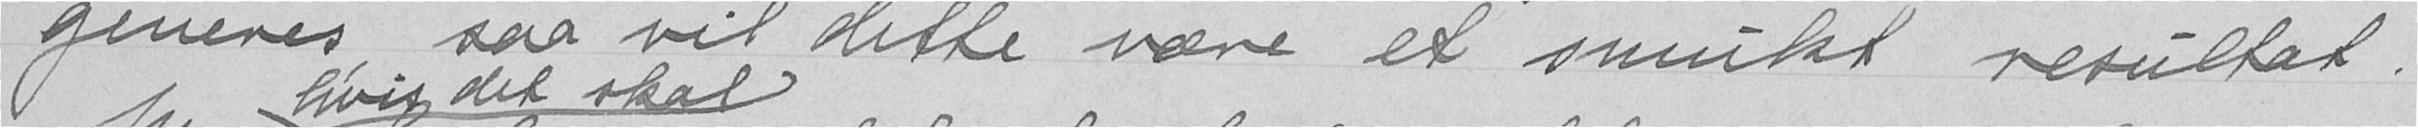generes saa vil dette være et smukt resultat.
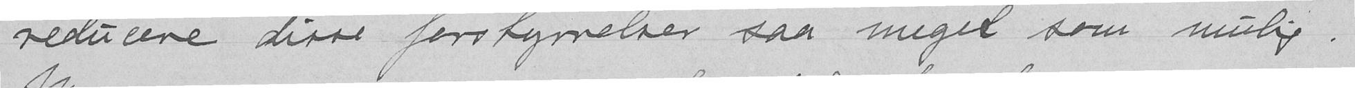reducere disse forstyrrelser saa meget som mulig.
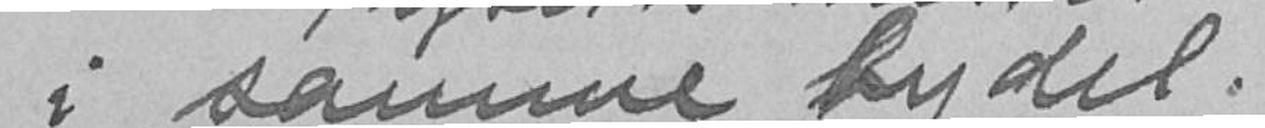i samme bydel.
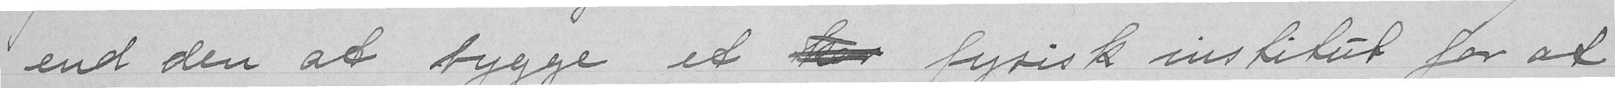end den at bygge et ke fysisk institut for at
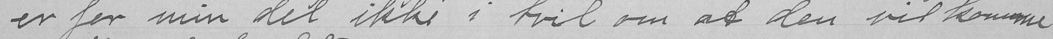er for min del ikke i tvil om at den vil komme
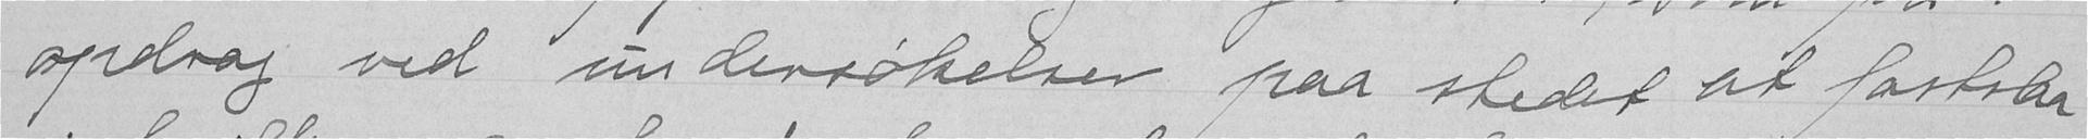opdrag ved undersøkelser paa stedet at fastslaa
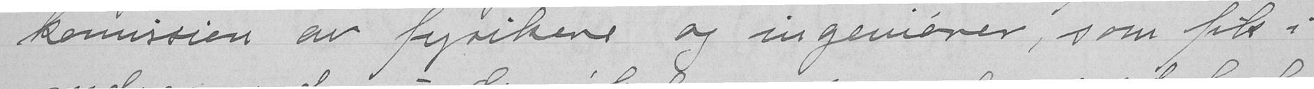komission av fysikere og ingeniører, som fik i
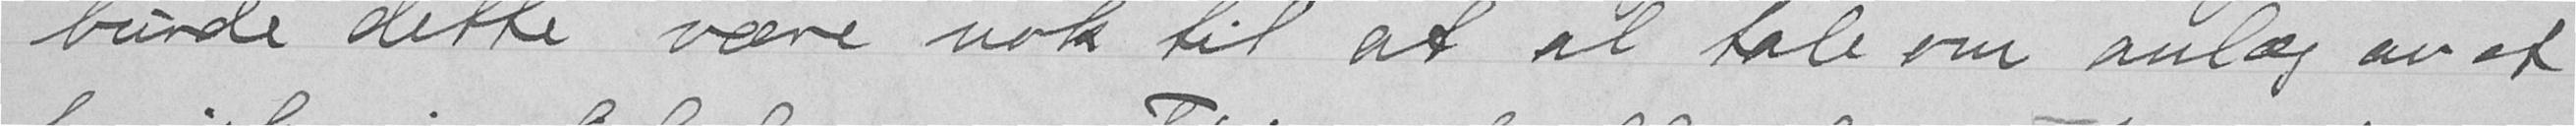burde dette være nok til at at tale om anlæg av et
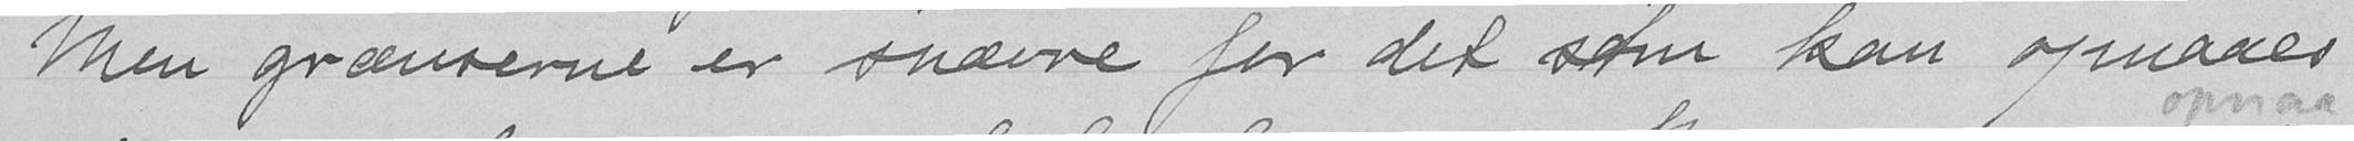Men grænserne er snævre for det som kan opnaaes
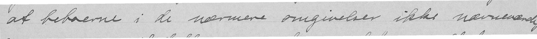at beboerne i de nærmere omgivelser ikke nævneværdig
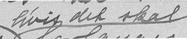hvis det skal
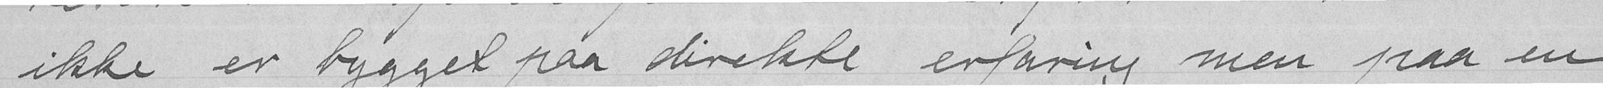ikke er bygget paa direkte erfaring men paa en
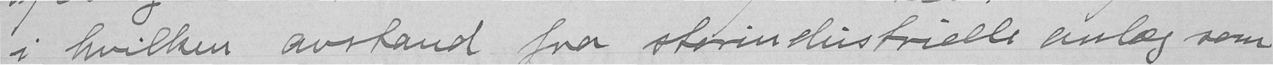i hvilken avstand fra storindustrielle anlæg som
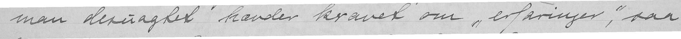man deruagtet hævder kravet om "erfaringer", saa
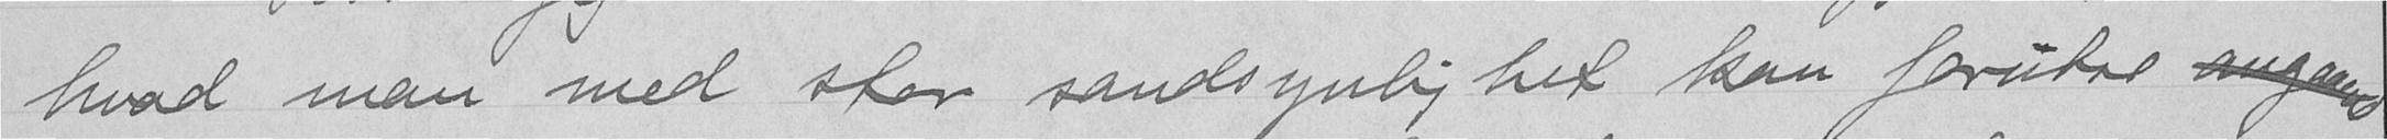hvad man med stor sandsynlighet kan forutse angaaend
